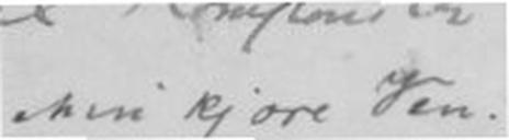Min kjære Ven.
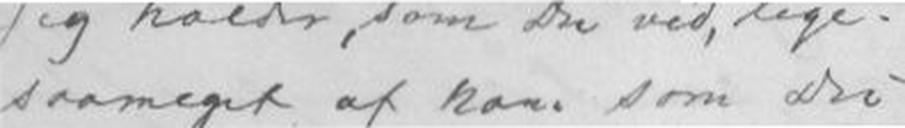saameget af ham som Du af
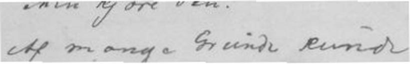Af mange Grunde kundej
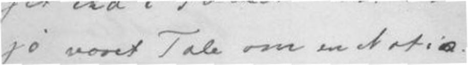jo været Tale om en Natio-
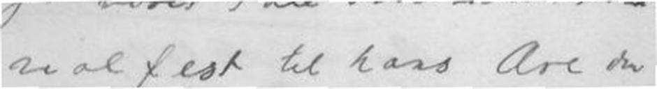nalfest til hans Ære den
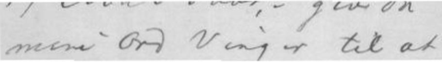mine Ord Vinger til at
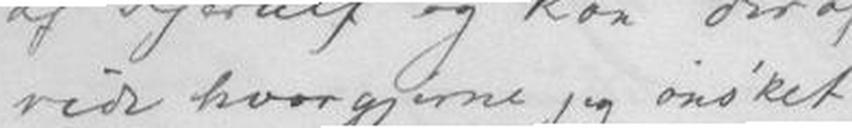vide hvor gjerne jeg ønsket
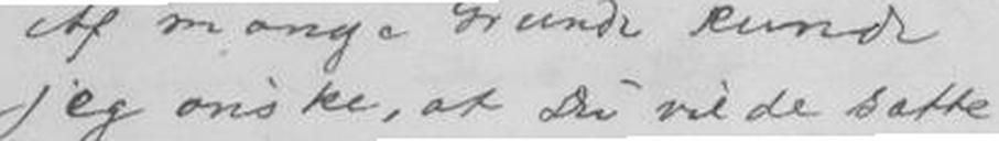eg ønsker, at Du vilde sætte
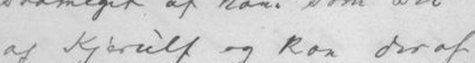Kjærulf og kan deraf
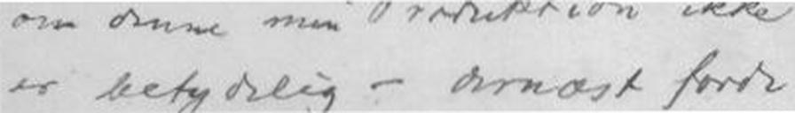er betydelig - dernæst fordi
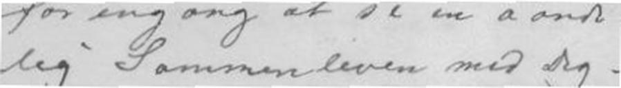lig Sammenleven med dig -
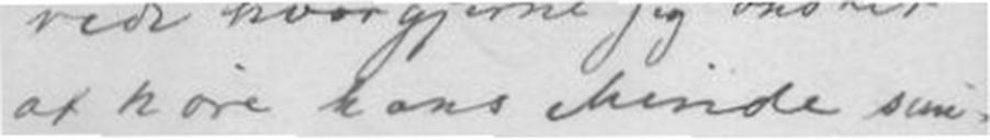at høre hans Minde sun-
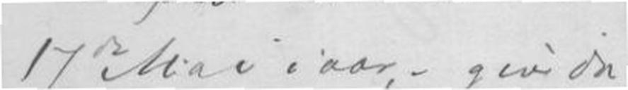17de Mai iaar, - give der
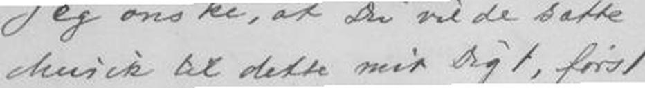Musik til dette mit digt, først
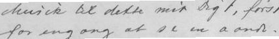for engang at se en aande-
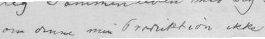om denne min Produktion ikke
